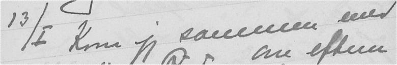13/I Kom jeg sammen med
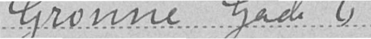Grønne Gade 6
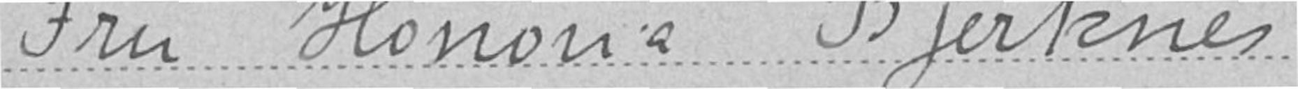Fru Honoria Bjerknes
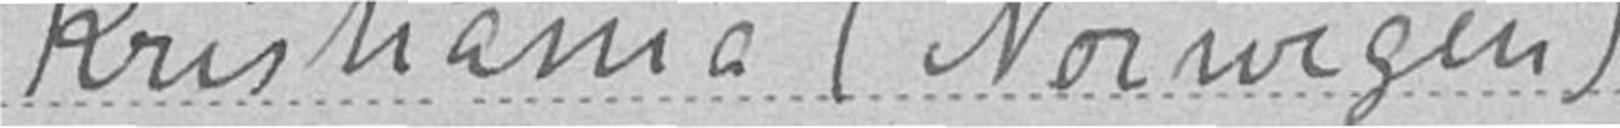Kristiania (Norwegen)
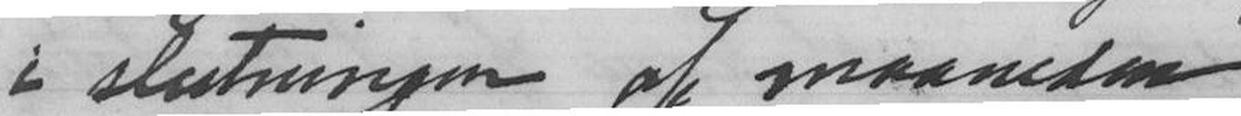i slutningen af maaneden
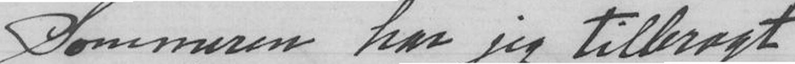Sommeren har jeg tilbragt
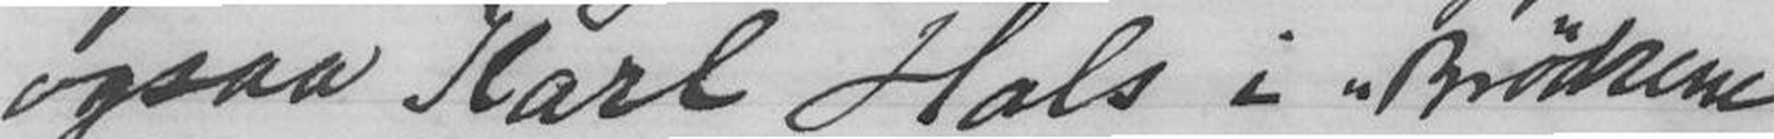ogsaa Karl Hals i Brødrene
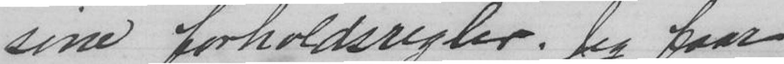sine forholdsregler. Jeg faar
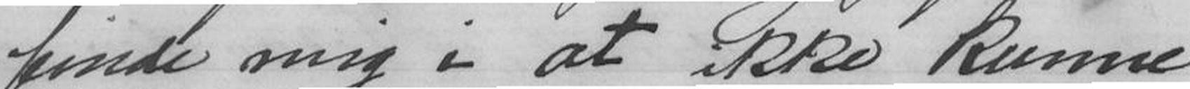finde mig i at ikke kunne
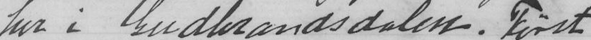her i Gudbrandsdalen. Først
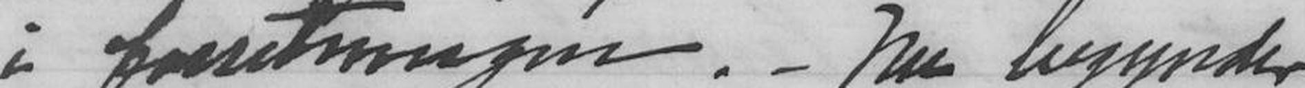i forretningen. - Nu begynder
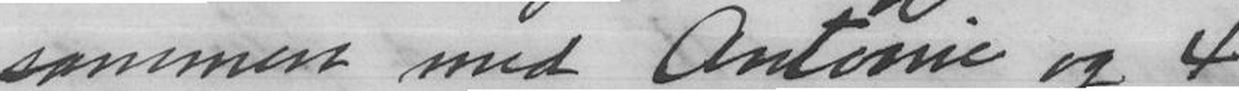sammen med Antonie og 4
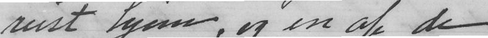reist hjem, og en af de
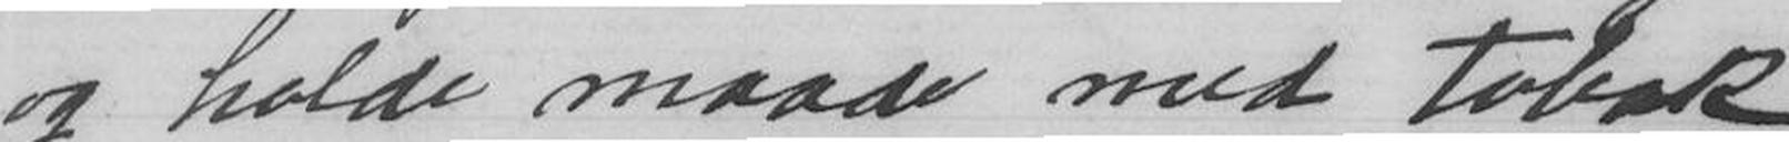og holde maade med tobak.
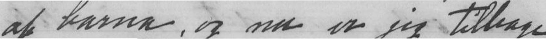af barna, og nu er jeg tilbage
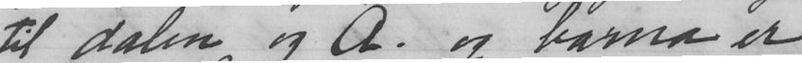til dalen og A. og barna er
